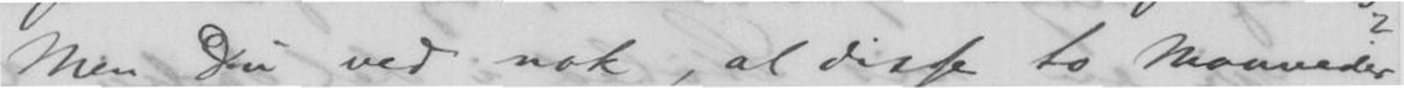Men Du ved nok, at disse to Maaneder
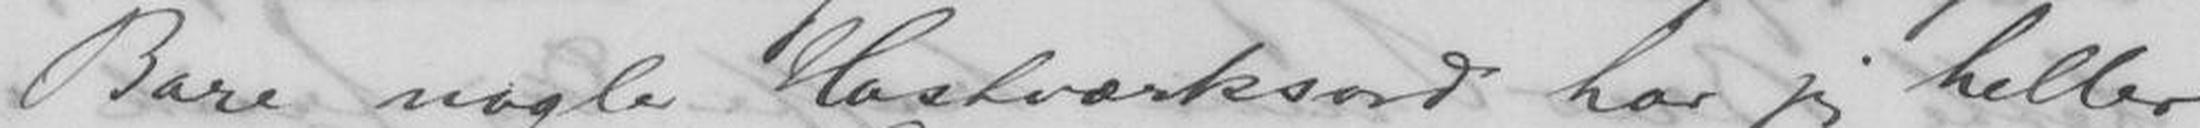osv. Bare nogle Hastværksord har jeg heller
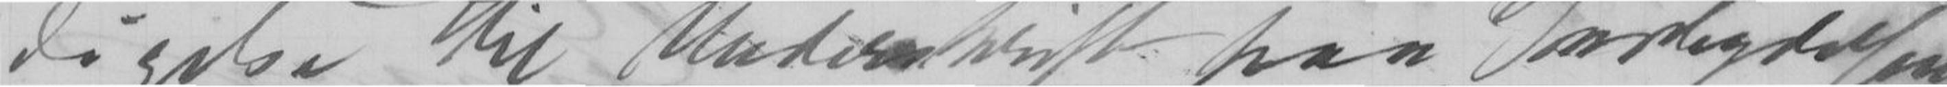digelse til Underskrift paa Indbydelsen.
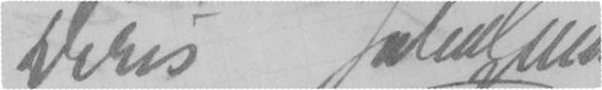Deres John Lund
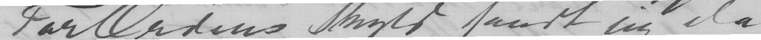For Ordens skyld fandt jeg da
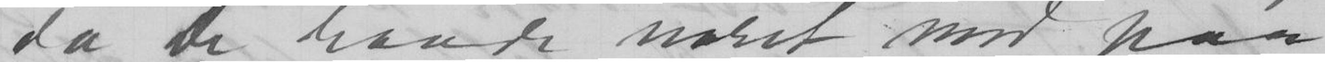da De havde været med paa
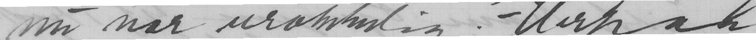nu var urokkelig. Herpaa
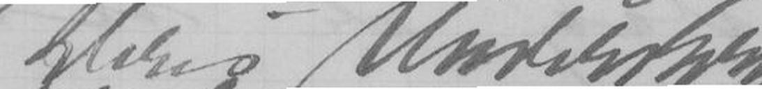Deres Underskrift.
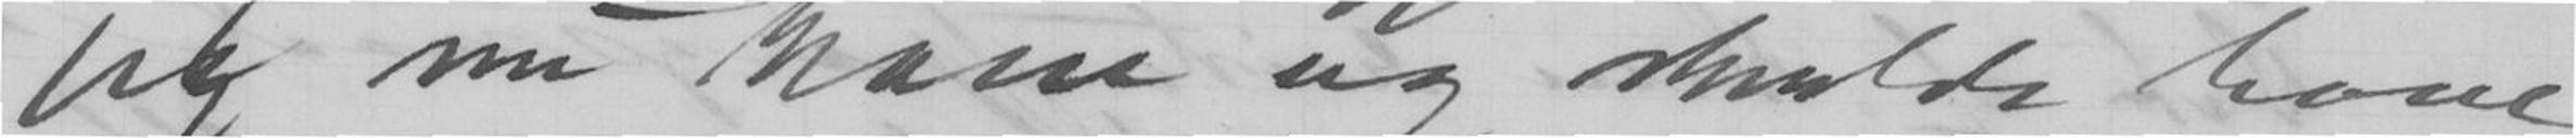jeg nu kom og skulde have
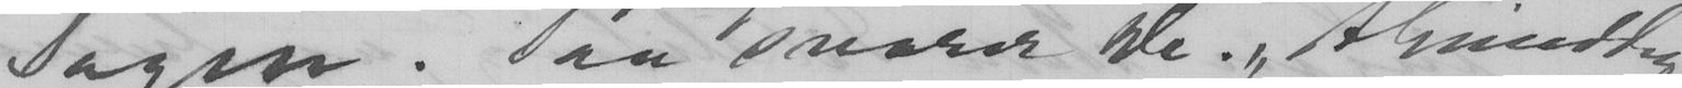Sagen. Saa svarer De. Almindlig
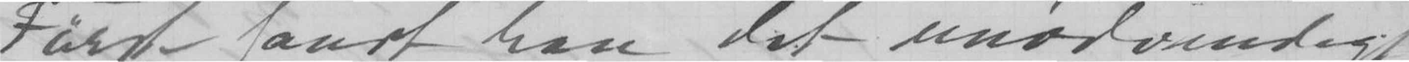Først fandt han det unødvendigt
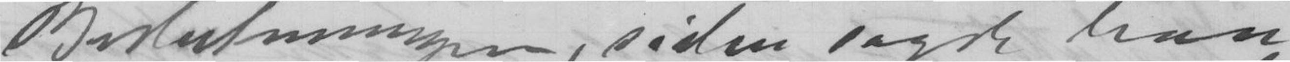Beslutningen, siden sagde han
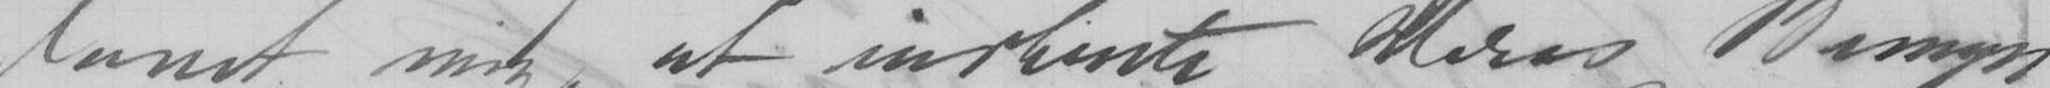lovet mig at indhente Deres Bemyn
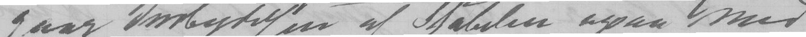gaar Indbydelsen af Stabelen ogsaa med
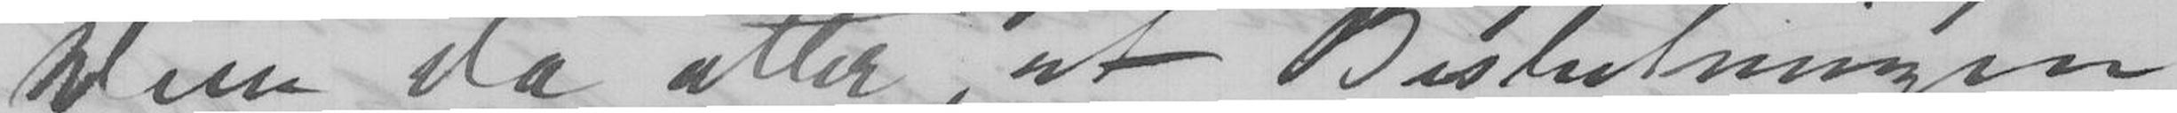Dem da atter, at Beslutningen
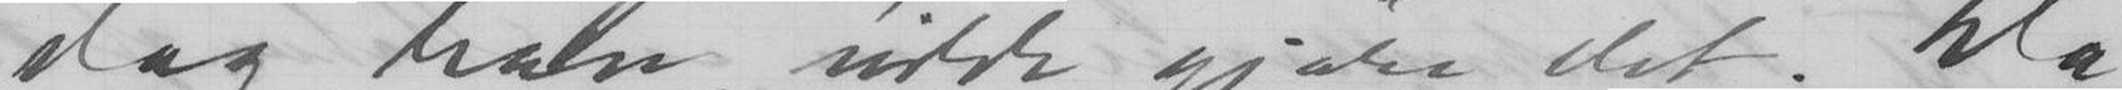dog han vilde gjøre det. Da
